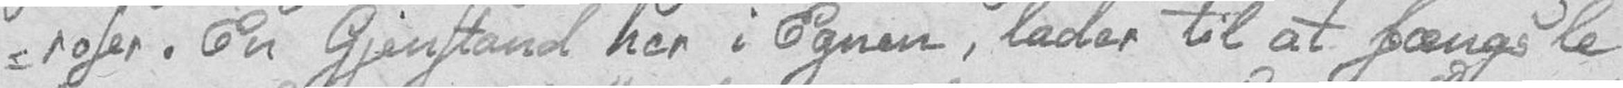-roser. En Gjenstand her i Egnen, lader til at fængsle
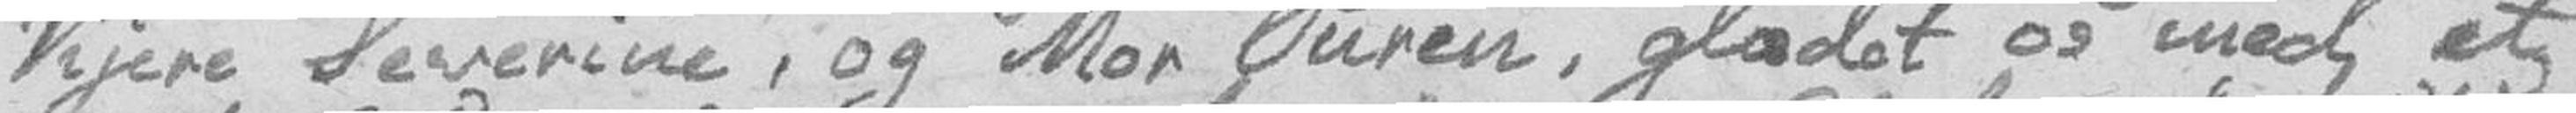kjere Severine, og Mor Ouren, glædet os med et
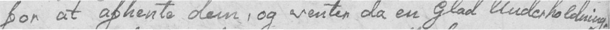for at afhente dem, og venter da en Glad Underholdning.
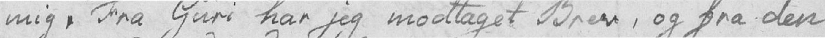mig. Fra Guri har jeg modtaget Brev, og fra den
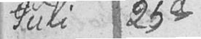Juli 25d
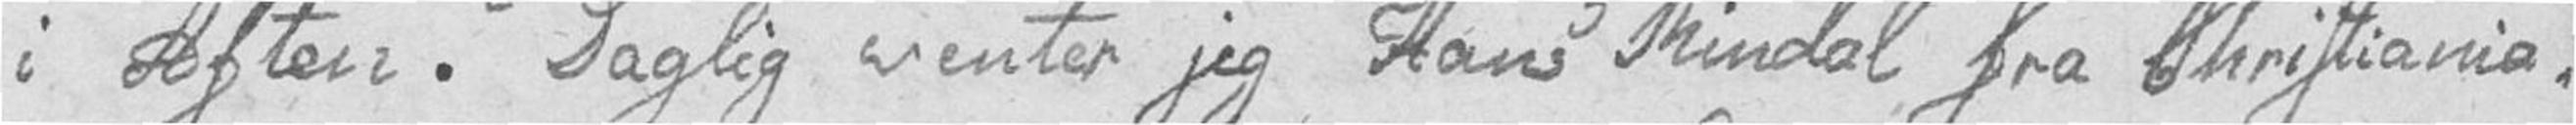i Aften. Daglig venter jeg Hans Rindal fra Christiania,
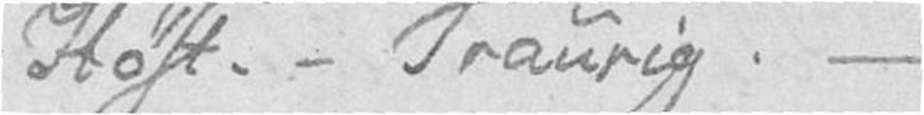Høst. – Traurig. –
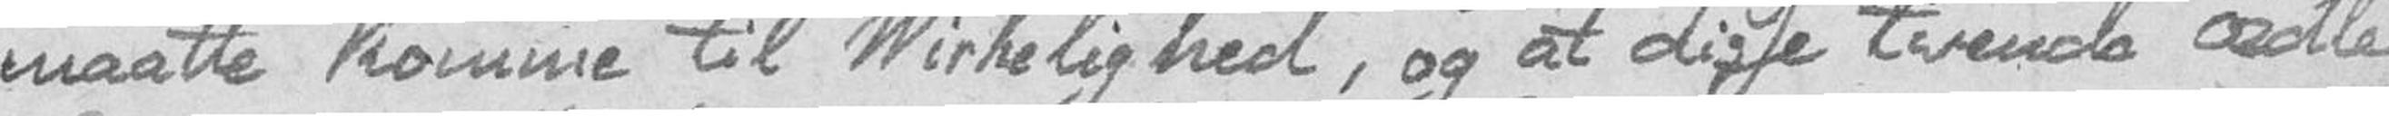maatte komme til Virkelighed, og at disse tvende ædle
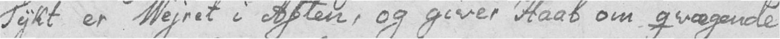Tykt er Vejret i Aften, og giver Haab om qvægende
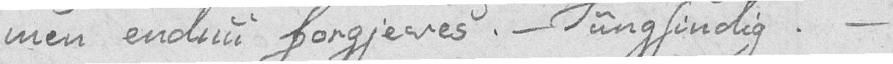men endnu forgjeves. – Tungsindig. –
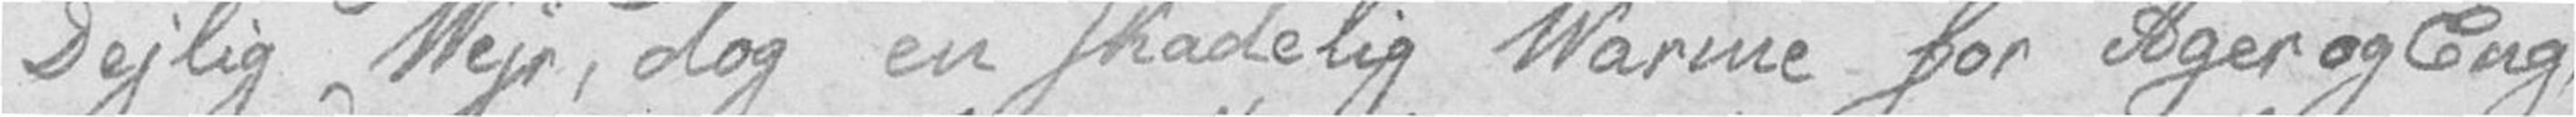Dejlig Vejr, dog en skadelig Varme for Ager og Eng,
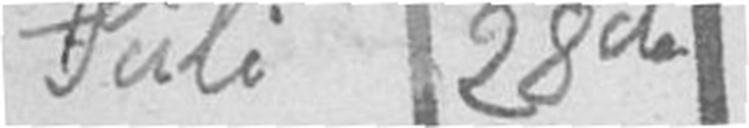Juli 28de
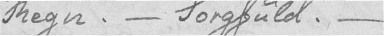Regn. – Sorgfuld. –
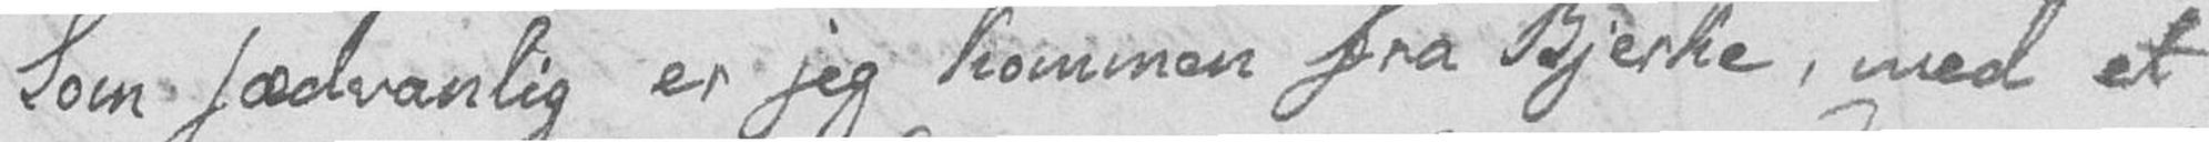Som sædvanlig er jeg kommen fra Bjerke, med et
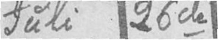Juli 26de
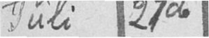Juli 27de
